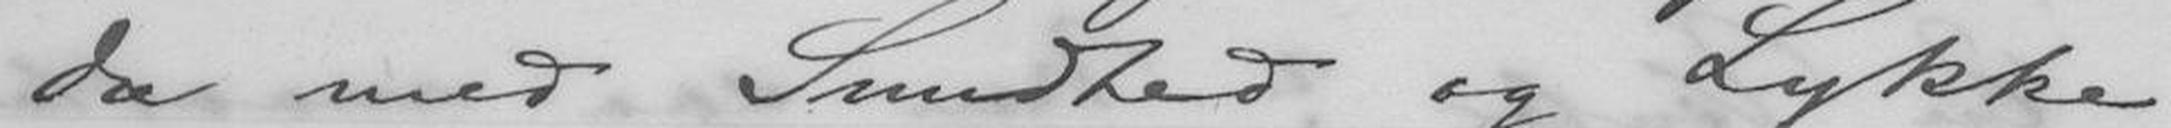da med Sundhed og Lykke
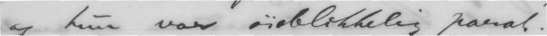og hun var øieblikkelig parat.
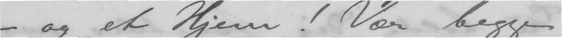- og et Hjem! Vær begge
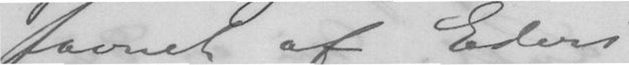favnet af Eders
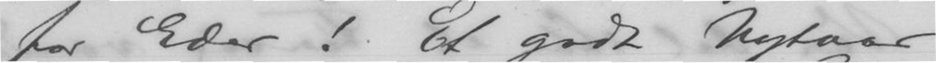for Eder! Et godt Nytaar
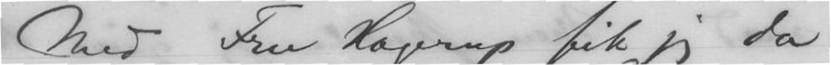Med Fru Hagerup fik jeg da
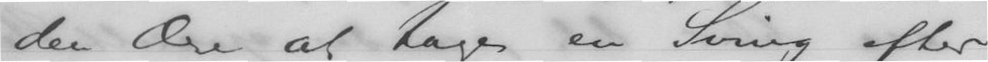den Ære at tage en Sving efter
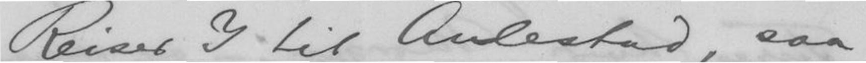Reiser I til Aulestad, saa
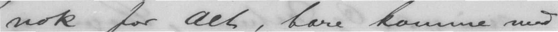nok for Alt, bare komme med
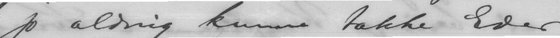jo aldrig kunne takke Eder
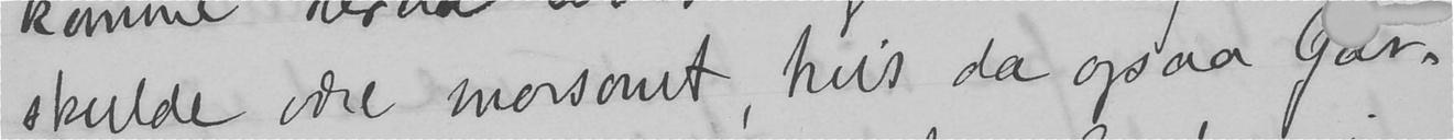skulde være morsomt, hvis da ogsaa Gar-
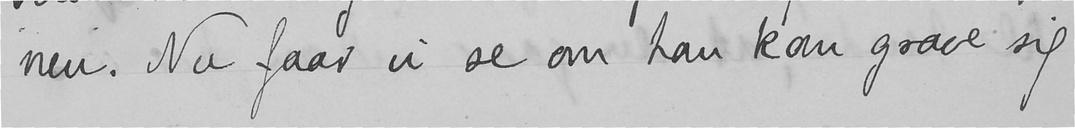nen. Nu faar vi se om han kan grave sig
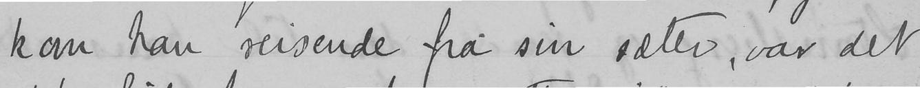kom han reisende fra sin sæter, var det
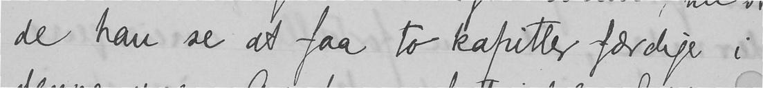de han se at faa to kapitler færdige i
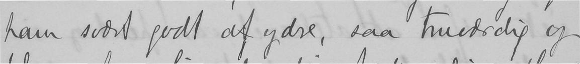ham svært godt af ydre, saa truværdig og
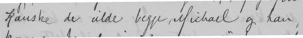Kanske de vilde begge, Michael og han,
